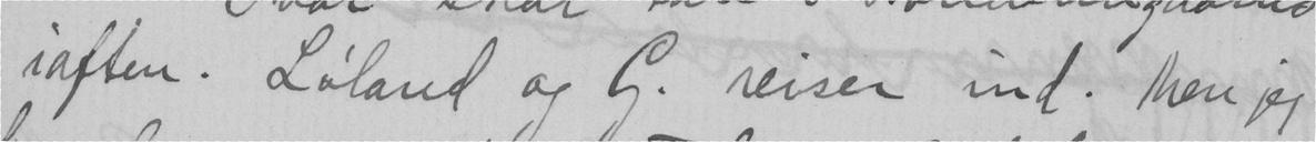iaften. Løland og G. reiser ind. Men jeg
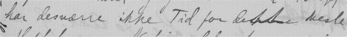har desværre ikke Tid for dette vesle
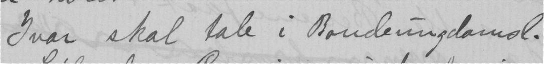Ivar skal tale i Bondeungdomsl.
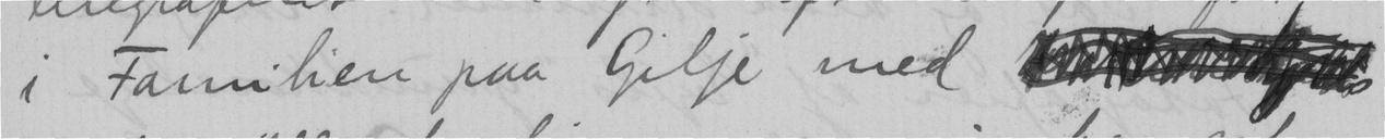i Familien paa Gilje med
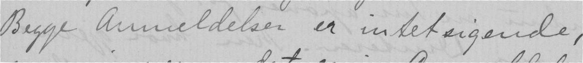Begge Anmeldelser er intetsigende,
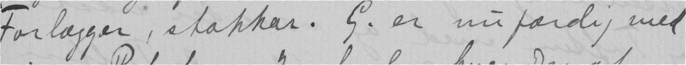Forlægger, stakkar. G. er nu færdig med
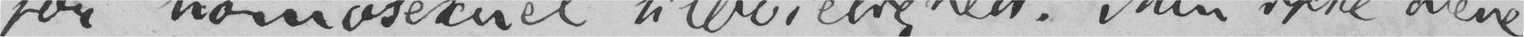for homosexuel tilbøielighed. Min ikke alene
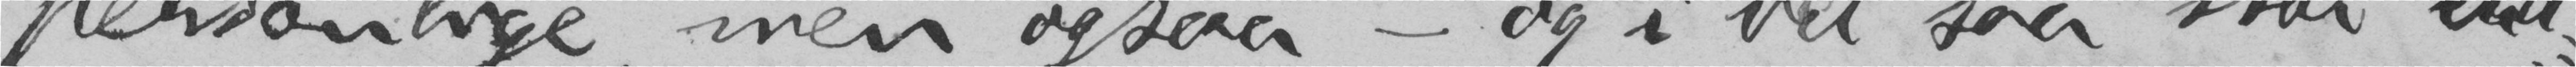personlige, men ogsaa - og i vel saa stor ud-
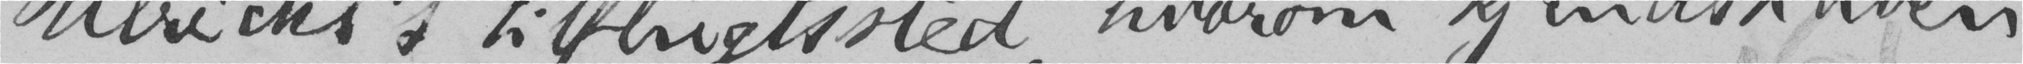Ulrichs’s tilflugtssted, hvorom kjendskaben
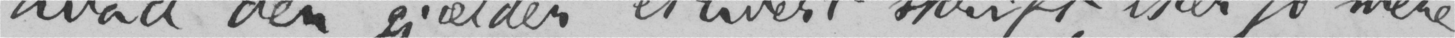hvad der gjælder ethvert skrift, især jo mere
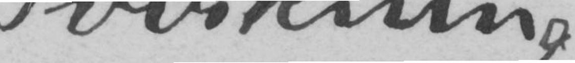virkning,
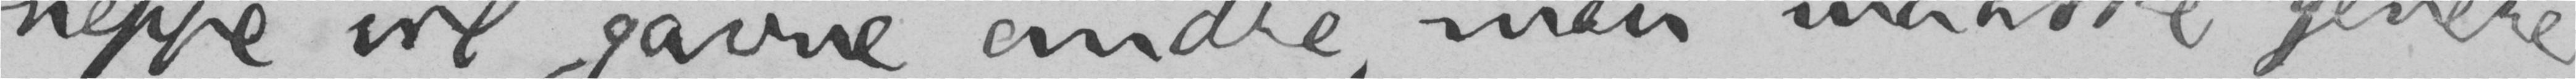neppe vil gavne andre, men maaske genere
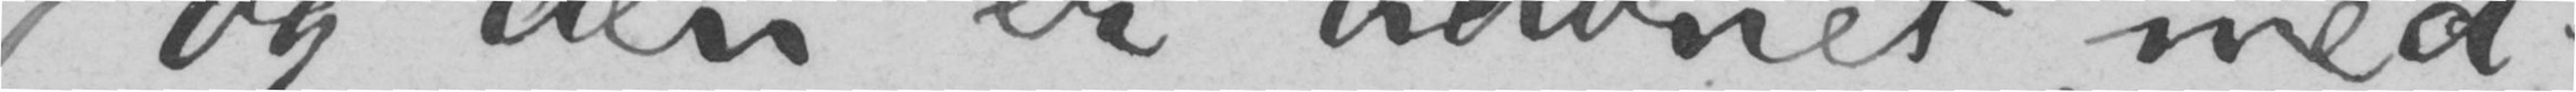, og den er aabnet med
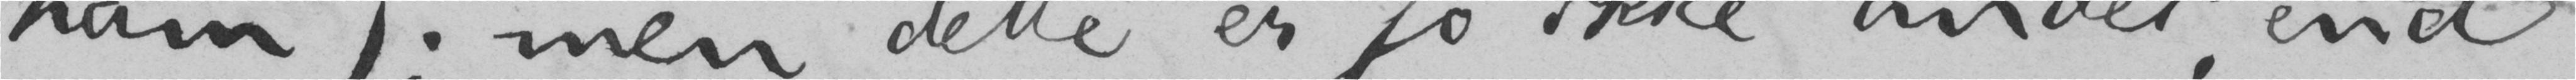ham); men dette er jo ikke andet, end
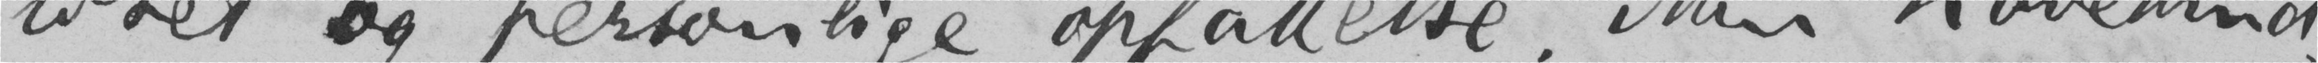litet og personlige opfattelse. Min hovedind-
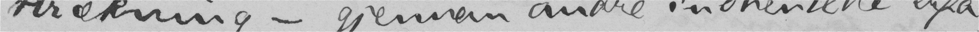strækning - gjennem andre indhentede erfa-
